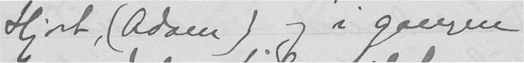Hjort, (Adam) og i gangen
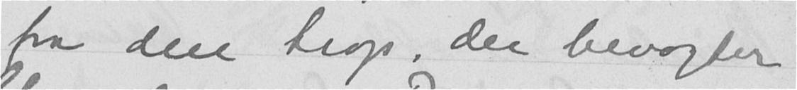fra den trop, der bevogter
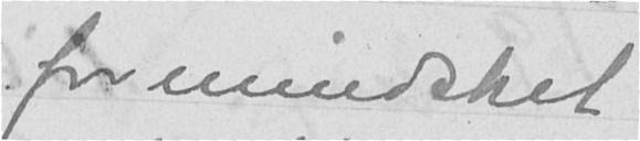formindsket
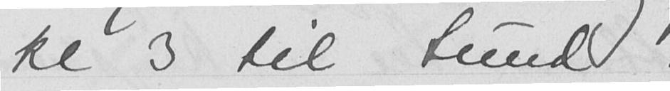kl 3 til Sund.
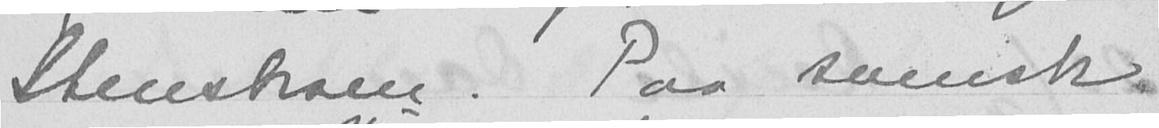Stensbroen. Paa svensk
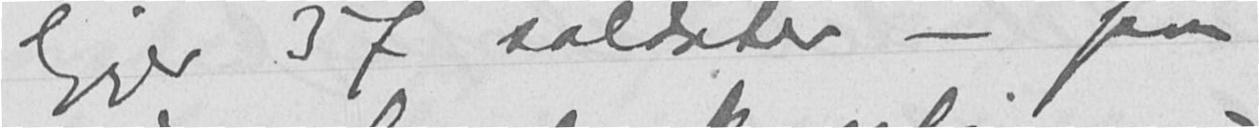ligger 37 soldater - paa
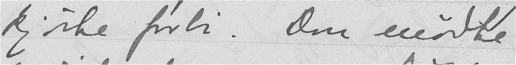kjørte forbi. Don mødte
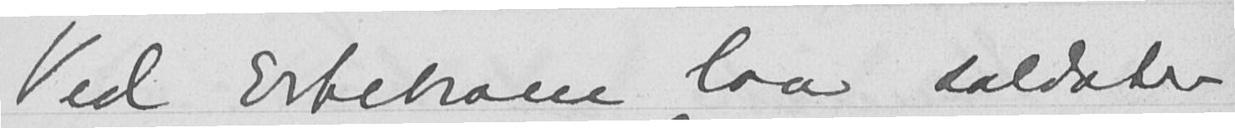Ved Ertebroen laa soldater
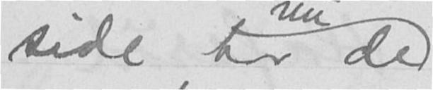side har de
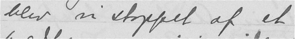blev vi stoppet af et
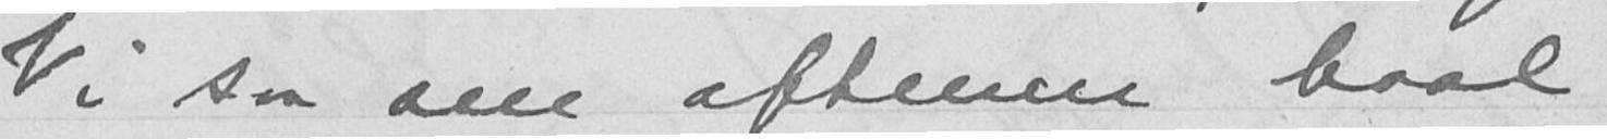Vi saa om aftenen baal
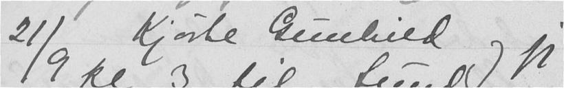21/9 Kjørte Gunhild og jeg
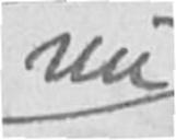nu
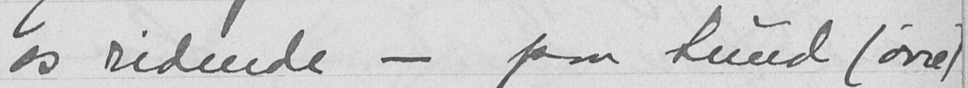os ridende - paa Sund (øvre)
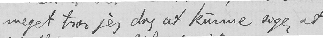meget tror jeg dog at kunne sige, at
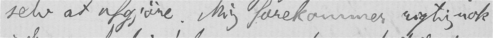selv at afgjøre. Mig forekommer rigtignok
